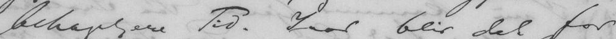behageligere Tid. Iaar blir det for
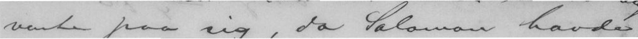vente paa sig, da Salomon havde
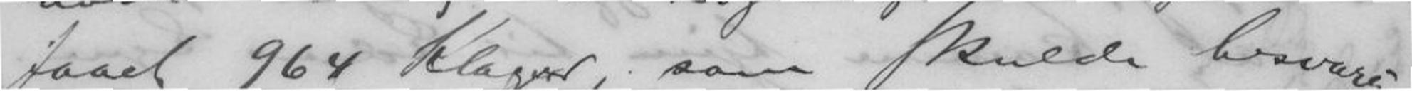faaet 964 Klager, som skulde besvares
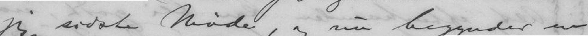jeg sidste Møde, og nu begynder en
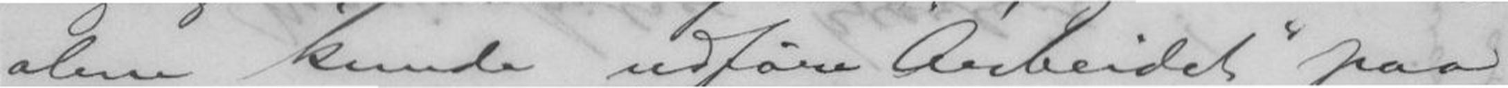alene kunde udføre Arbeidet "paa
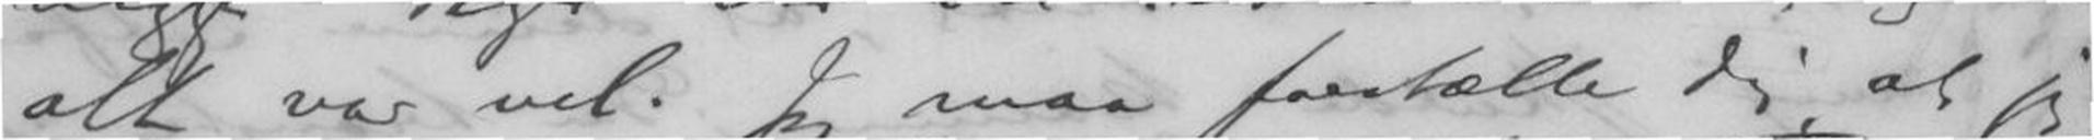alt var vel. Jeg maa fortælle dig, at jeg
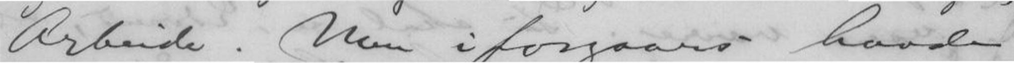Arbeide. Men iforgaars havde
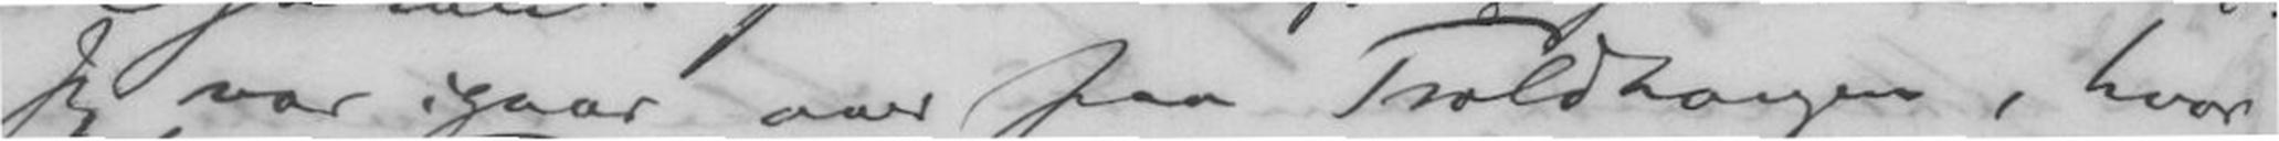Jeg var igaar over paa Troldhaugen, hvor
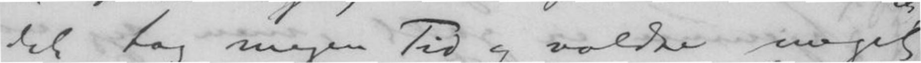og det tog megen Tid og voldte meget
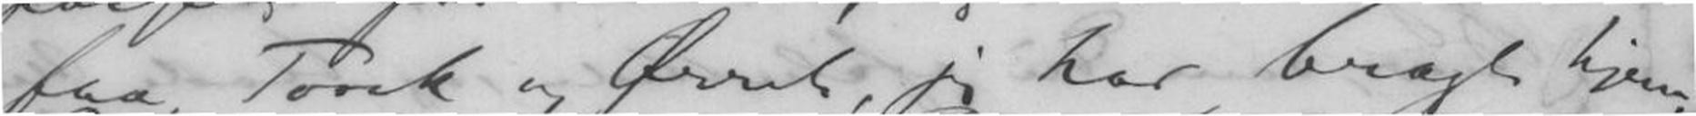faa Torsk og Ørret, jeg har bragt hjem
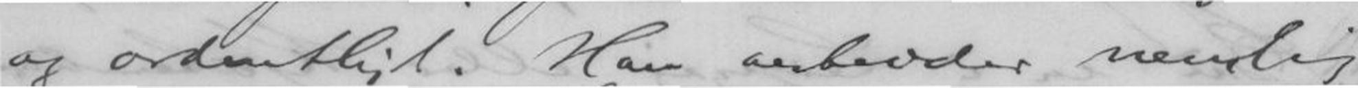og ordentligt. Han arbeider nemlig
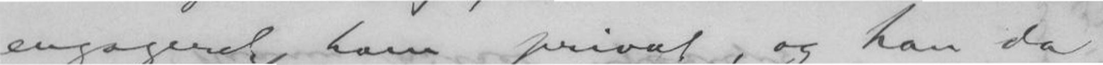engageret ham privat, og han da
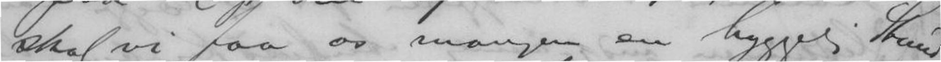skal vi faa os mangen en hyggelig Stund
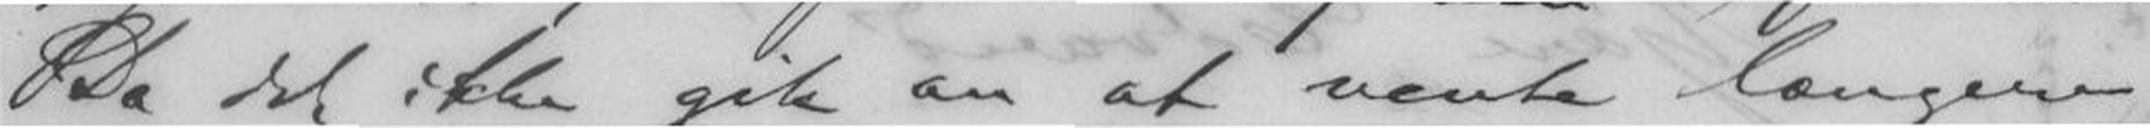Da det ikke gik an at vænte længere,
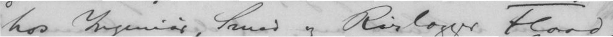hos Ingeniør, Smed og Rørlægger Flood,
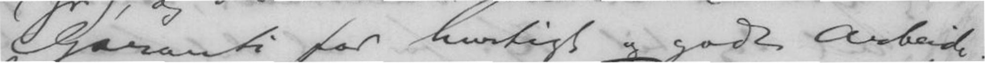Garanti for hurtigt og godt Arbeide.
